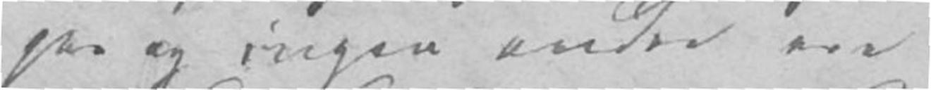ger og ingen andre ere
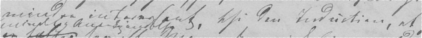mindre interessant, thi den Induction, at
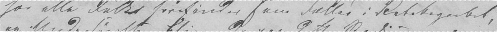for alle Folk forefinder som Fælles i Retsbegrebet,
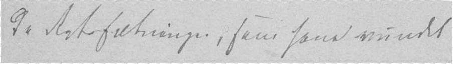de Retssætninger, som have vundet
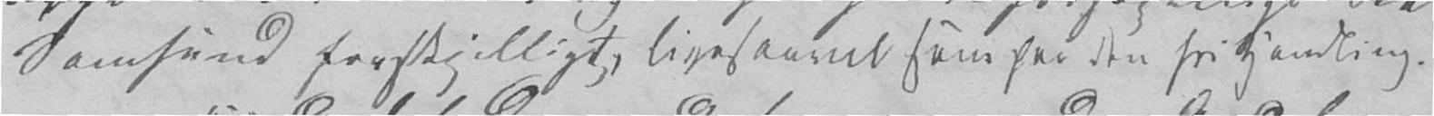Samfund forskjelligt, ligesaavel som paa den fri Handling.
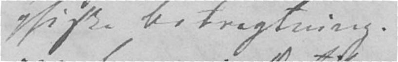phiske Betragtning.
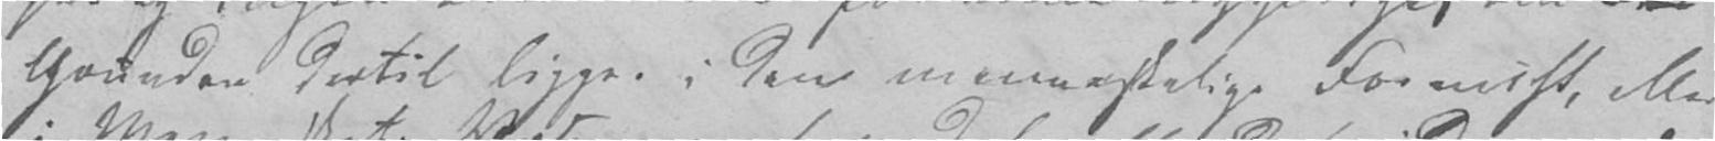Grunden dertil ligger i den menneskelige Fornuft, eller
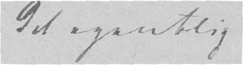det egentlig
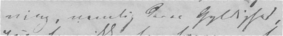ning, nemlig deres Gyldighed,
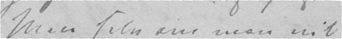Men selv om man vil
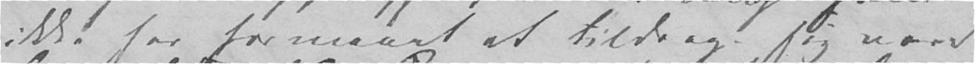ikke har formaaet at tildrage sig vore
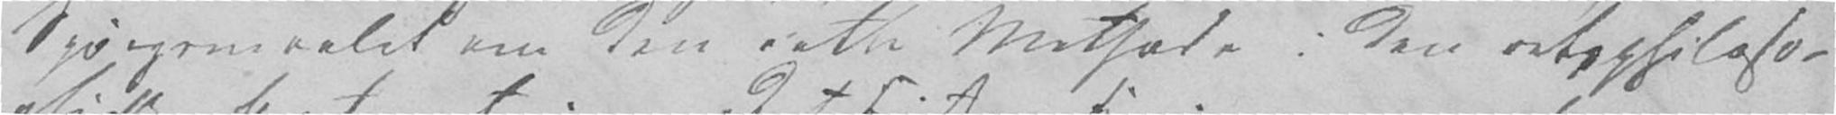Spørgsmaalet om den rette Methode i den retsphiloso-
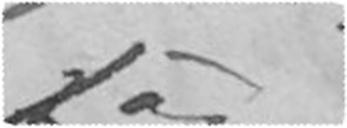som
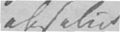absolut
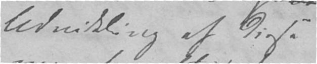Udvikling af disse
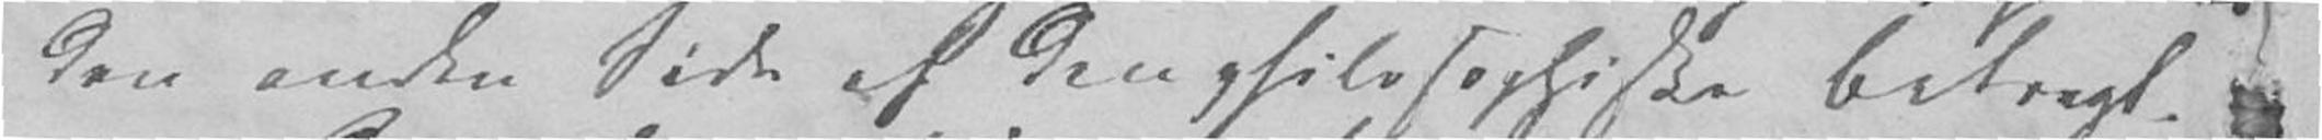den anden Side af den philosophiske Betragt-
